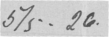5/5-26.
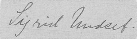Sigrid Undset.
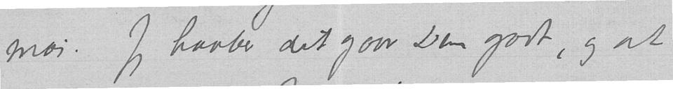mai. Jeg haaber det gaar Dem godt, og at
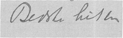Bedste hilsen
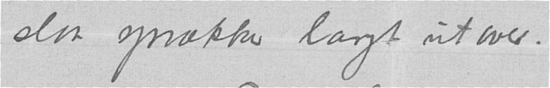slaa sprække langt utover.
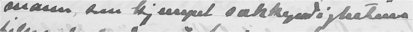mann, som kjempet sakkyndighetens
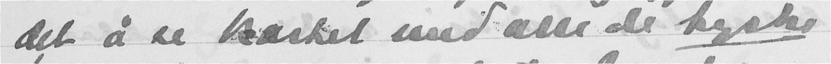det å se kortet med alle de tyske
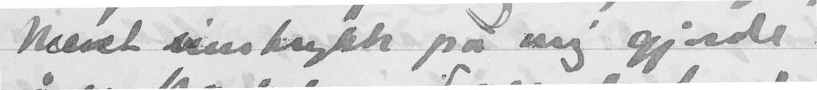Mest inntrykk på mig gjorde
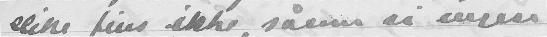slike fins ikke såsom vi ingen
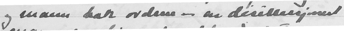og mann bak ordene - en desillusjonert
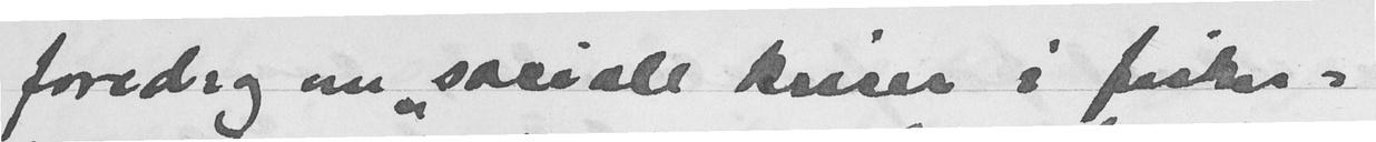foredag om "sociale kriser i fisker-
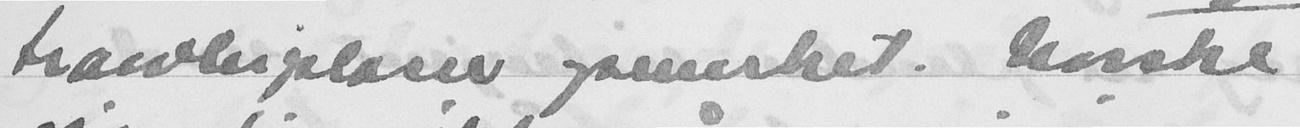havneplasser opmerket. Norske
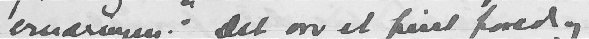inæringen." Det var et fint foredrag
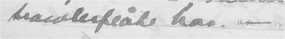broweles flåte har. -
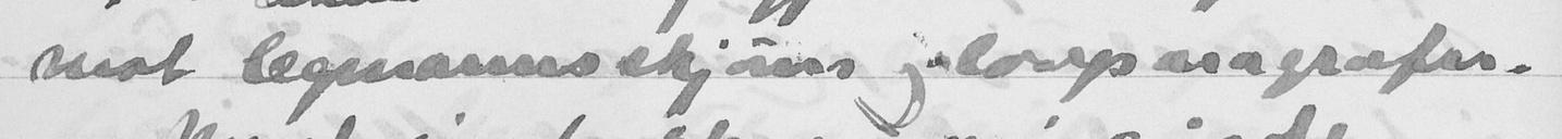mot legmanns skjønn og loipnsagrafir.
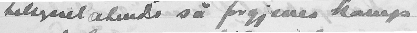tilsynelatende så forgjeves kamp
